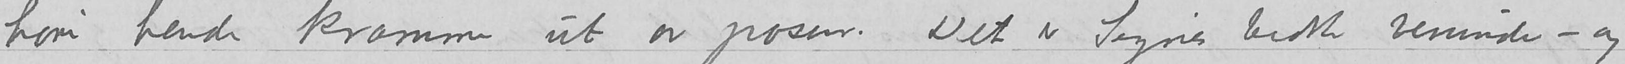høre hende kramme ut av posen. Det er Signes bedste veninde – og
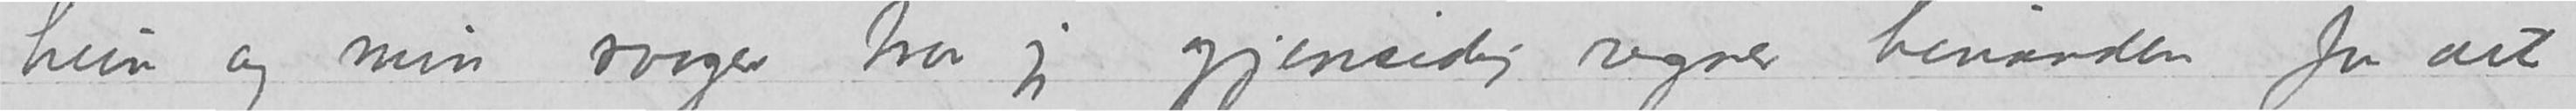hun og min svoger tror jeg gjensidig regner hinanden for det
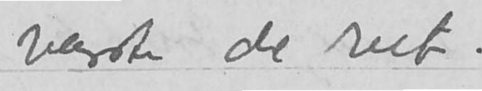værste de vet.
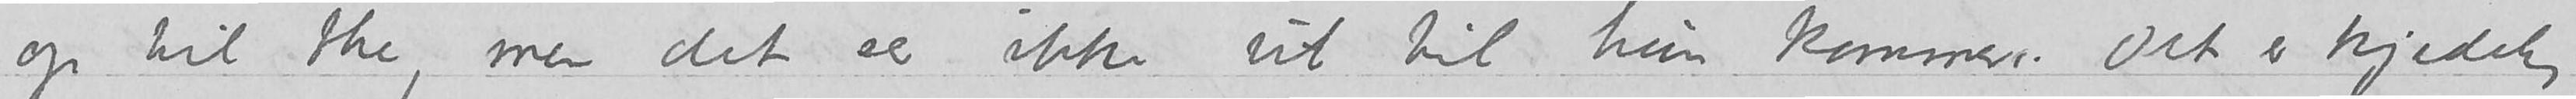op til the, men det ser ikke ut til hun kommer. Det er kjedelig
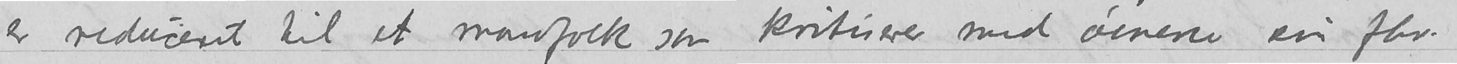er reduceret til et mandfolk som kritiserer med øinene sin fhr.
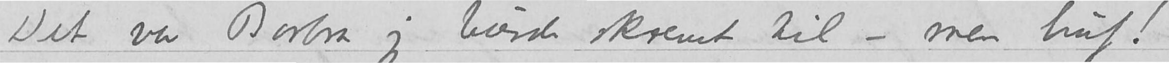Det var Barbra jeg burde skrevet til – men huf!
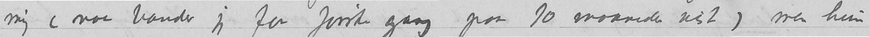mig (naa bander jeg for første gang paa 10 maaneder vist) men hun
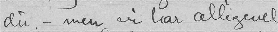du, - men vi har alligevel
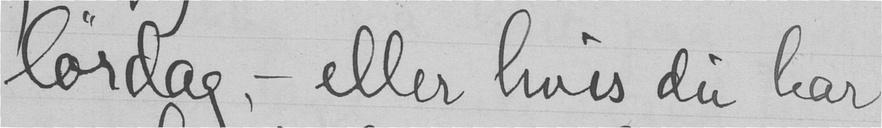lørdag, - eller hvis du har
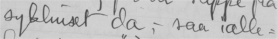sykehuset da, - saa ialle-
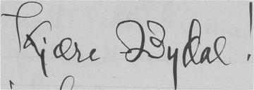Kjære Bydal!
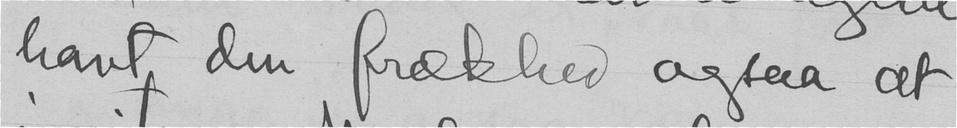havt den frækhed ogsaa at
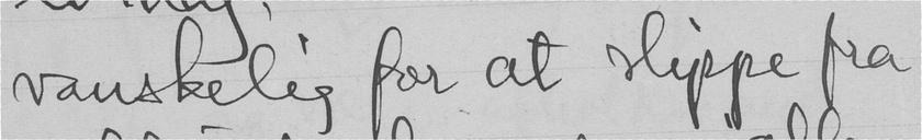vanskelig for at slippe fra
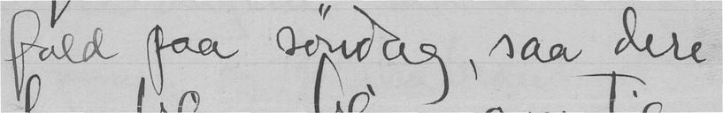fald paa søndag, saa dere
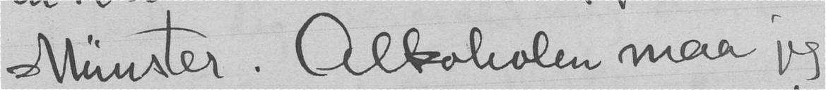Münster. Alkoholen maa jeg
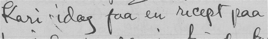Kari idag faa en recept paa
